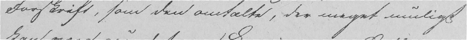Forskrift, som den omtalte, der meget muligt
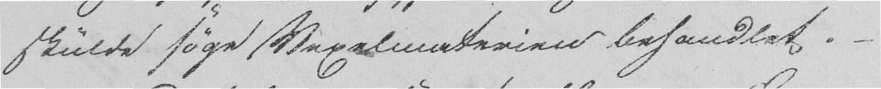skulde søge Vexelmaterien behandlet. -
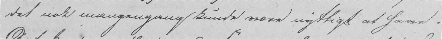det nok mangengang kunde være nyttigt at have.
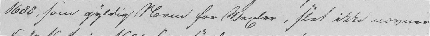1688, som gyldig Norm for Vexler, slet ikke nævner
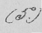(5.)
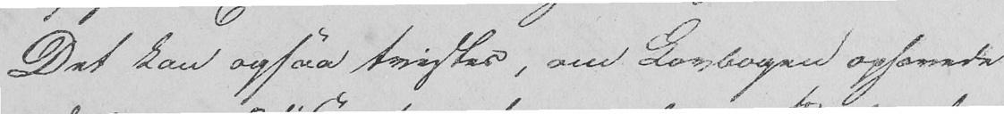Det kan ogsaa tvistes, om Lovbogen ophævede
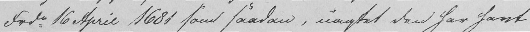Frdn 16 April 1681 som saadan, uagtet den har havt
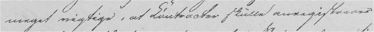meget vigtige, at Contracter skulle omregistreres
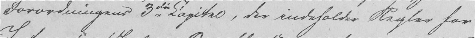Forordningens 3die Capitel, der indeholder Regler for
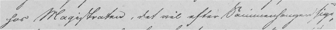hos Magistraten, det vil efter Sammenhængen sige,
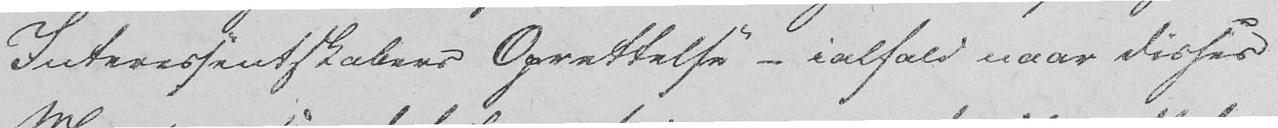Interessentskabers Oprettelse - ialfald naar disses
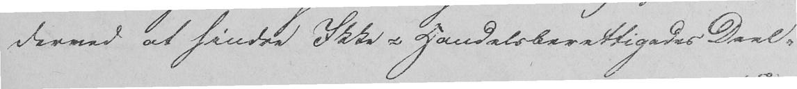derved at hindre Ikke-Handelsberettigedes Deel-
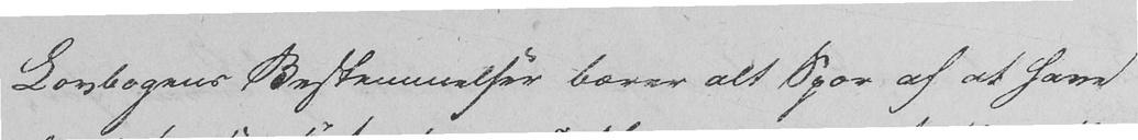Lovbogens Bestemmelser bærer alt Spor af at have
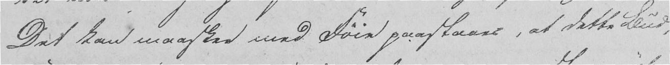Det kan maaskee med Føie paastaaes, at dette Bud,
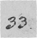33.
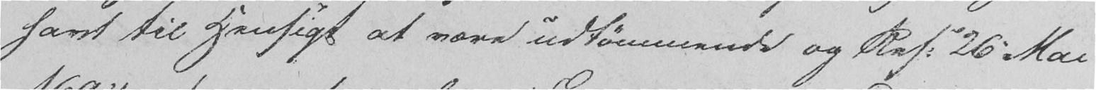havt til Hensigt at være udtømmende og Res. 26 Mai
